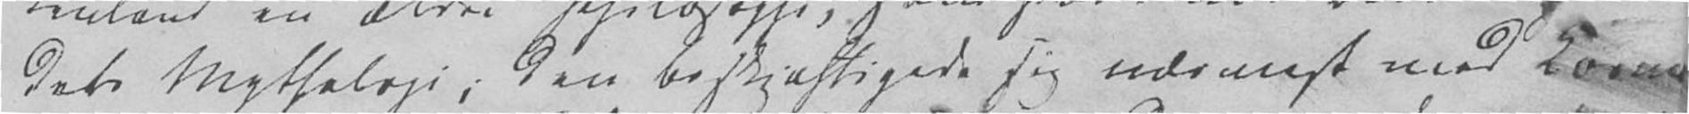dets Mythologi; den beskjeftigede sig nærmest med Kosmo-
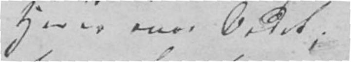Herre over Ordet;
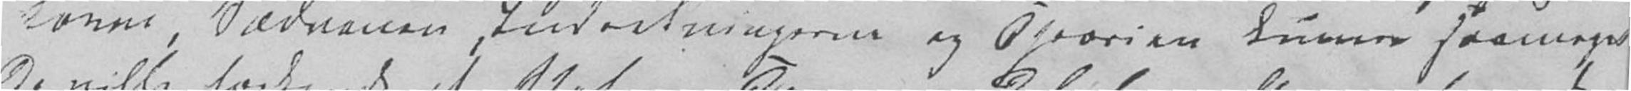Lovene, Sædvanen, Indretningerne og Theorien kunne saamegen
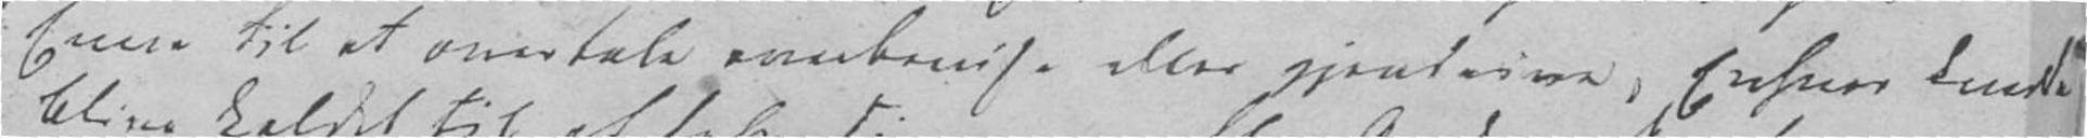Evne til at overtale overbevise eller gjendrive. Enhver kunde
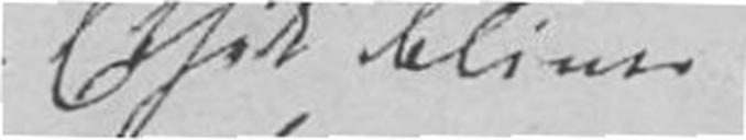Ethik bliver
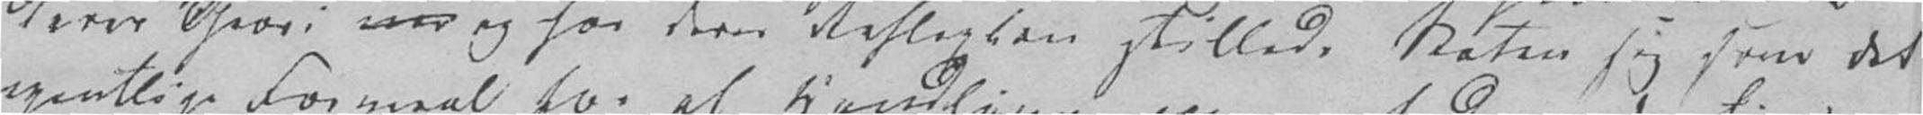deres Theori var og hos deres Reflexion stillede Staten sig som det
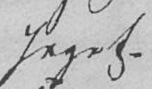Jeget.
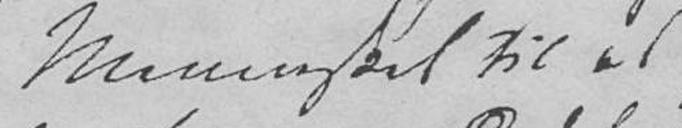Mennesket til at
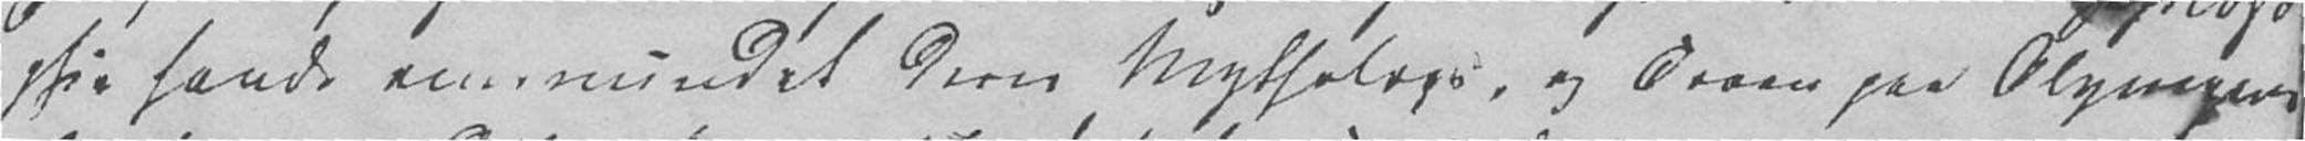phie havde overvundet deres Mythologie, og Troen paa Olympens
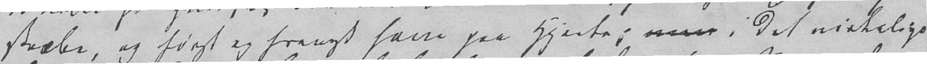stræbe, og først og fremst have paa Hjerte; men i det virkelige
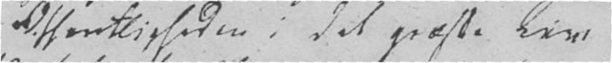Offentligheden i det græske Liv
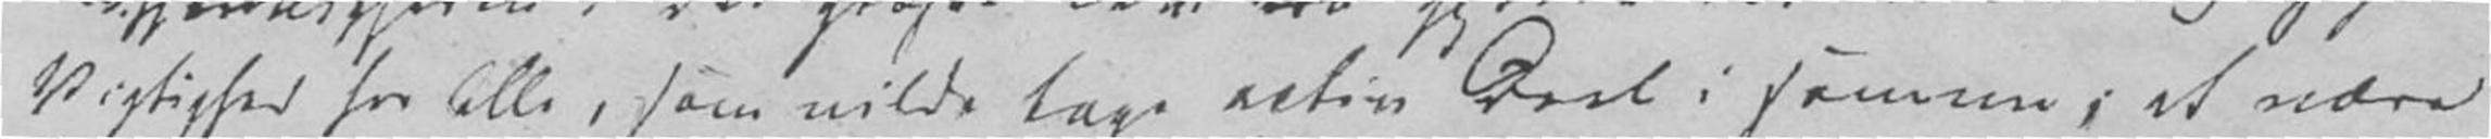Vigtighed for Alle, som vilde tage activ Deel i samme, at være
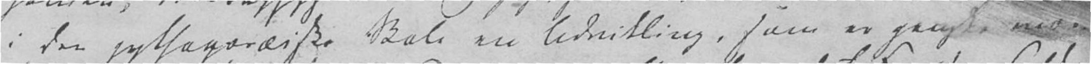i den pythagoræiske Skole en Udvikling, som er ganske mær-
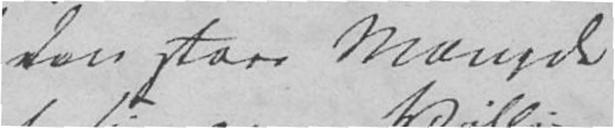af den store Mængde
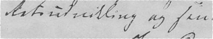Retsudvikling og sin
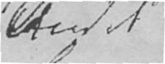Andet
